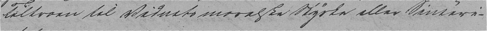Tiltroen til Vidnets moralske Styrke eller Sinceri-
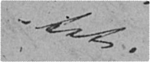tet.
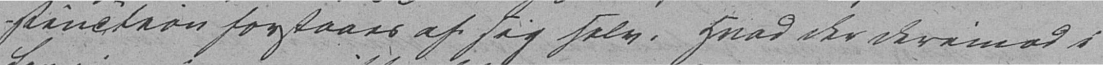stinction forstaaes af sig selv. Hvad der derimod i
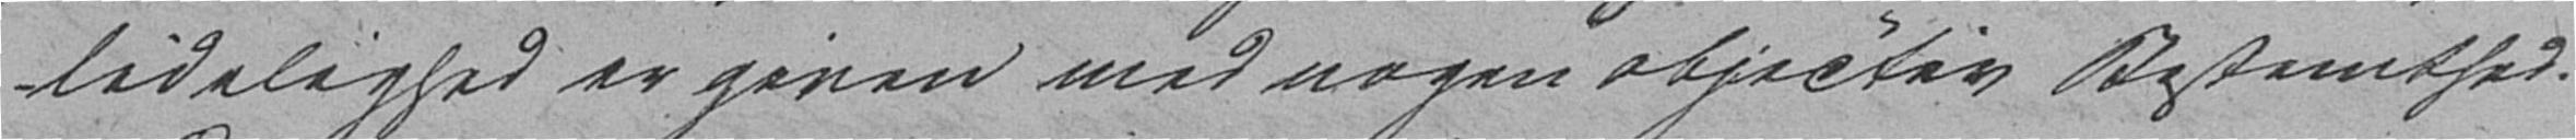lidelighed er given med nogen objectiv Bestemthed.
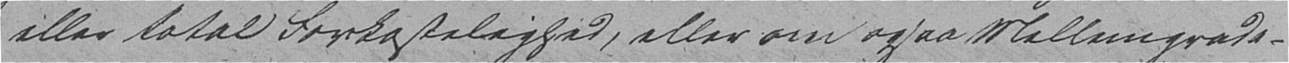eller total Forkastelighed, eller om ogsaa Mellemgrada-
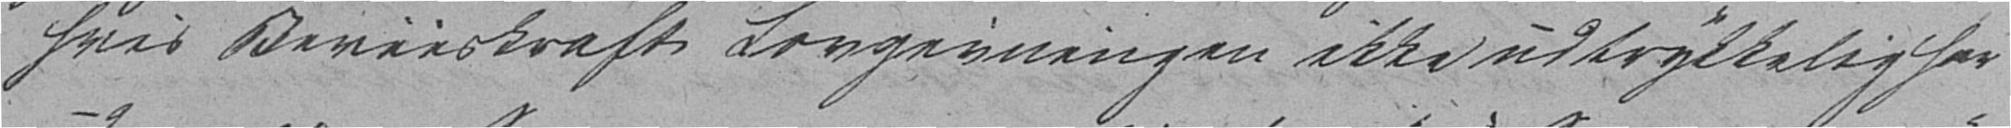hvis Beviiskraft Lovgivningen ikke udtrykkelig har
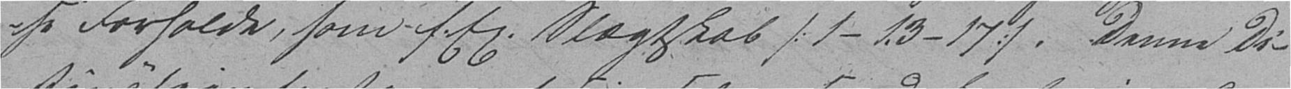se Forholde, som f. Ex. Slægtskab /: 1-13-17 :/. Denne Di-
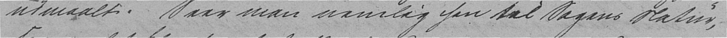udmaalt. Seer man nemlig hen til Sagens Natur,
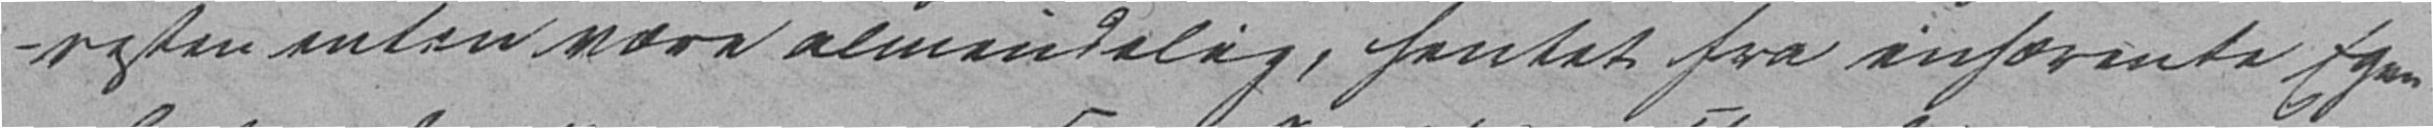resten enten være almindelig, hentet fra inhærente Egen-
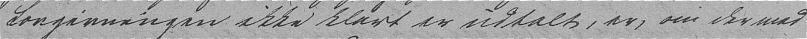Lovgivningen ikke klart er udtalt, er, om der med
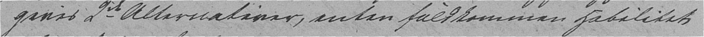gives 2de Alternativer, enten fuldkommen Habilitet
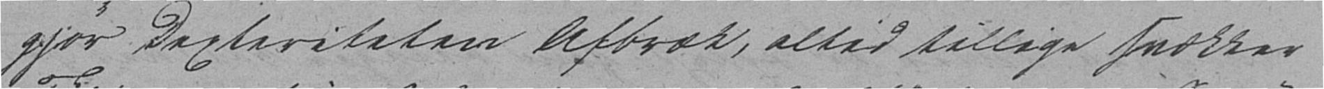gjør Dexteriteten Afbræk, altid tillige svækker
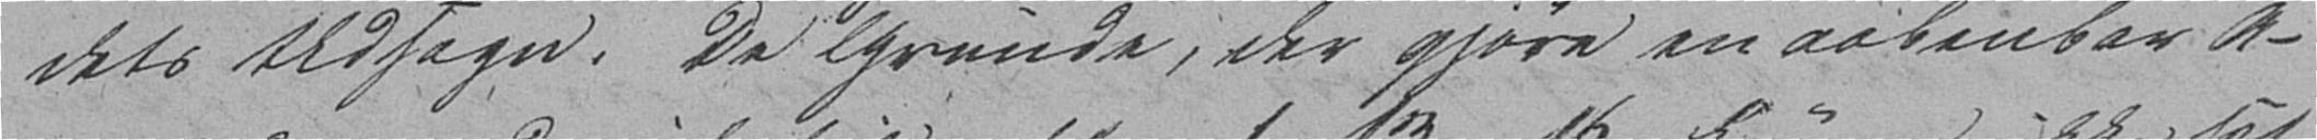dets Udsagn. De Grunde, der gjøre en aabenbar A-
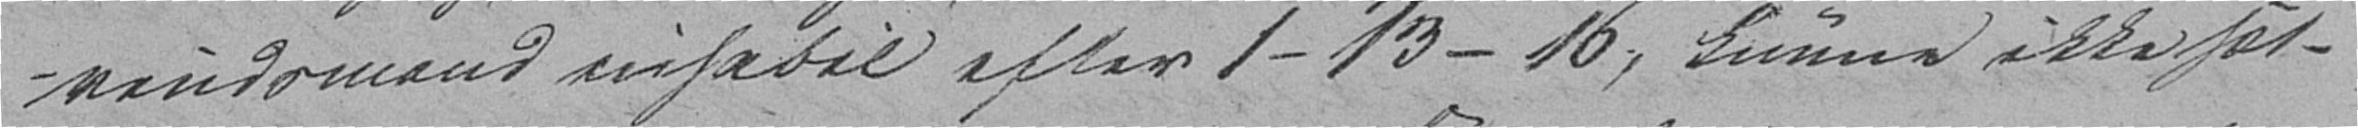vindsmand inhabil efter 1-13-16, kunne ikke sæt-
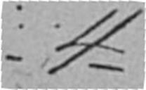- 4 -
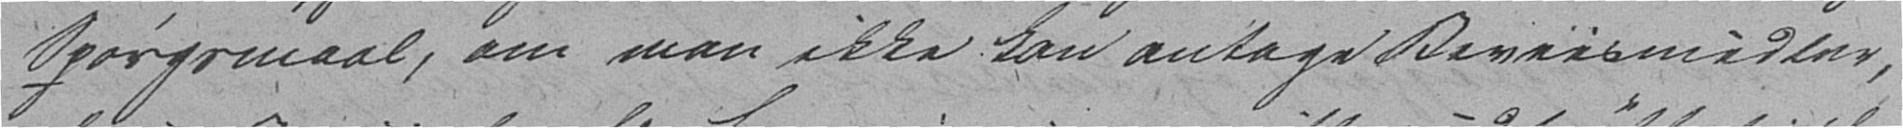Spørgsmaal, om man ikke kan antage Beviismidler,
